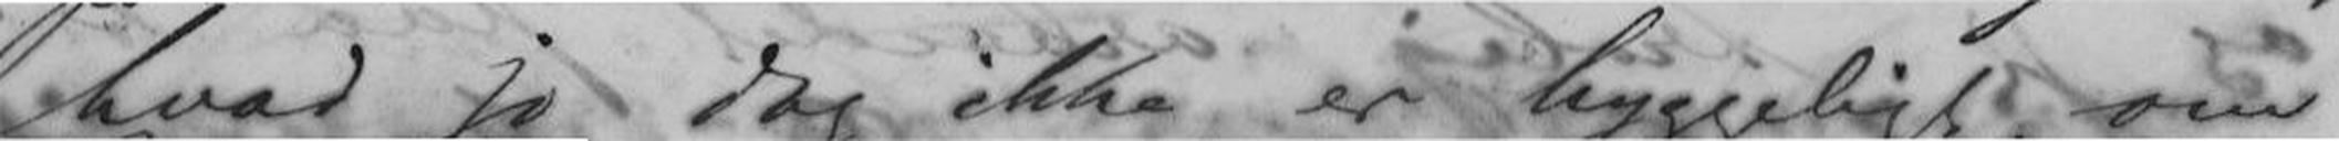hvad jo dog ikke er hyggeligt, om
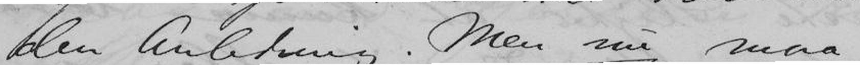den Anledning. Men nu maa jeg
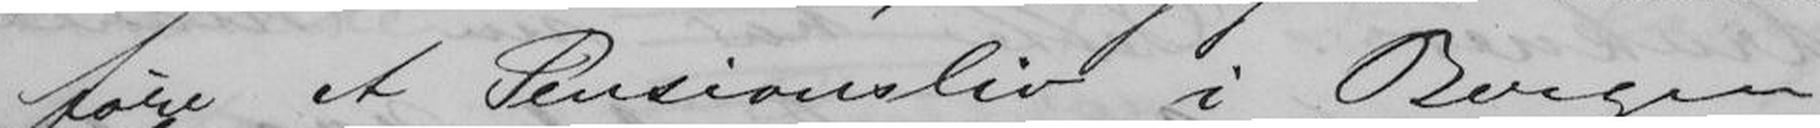føre et Pensionsliv i Bergen,
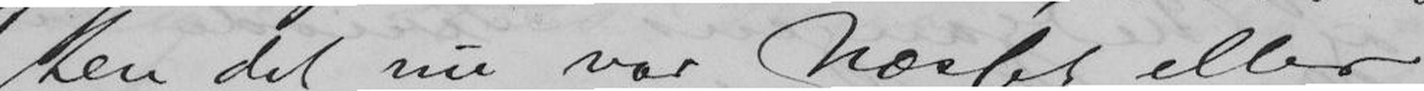ten det nu var Næsset eller
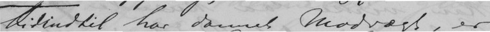didindtil har dannet Modvægt, er
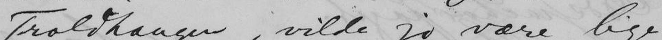Troldhaugen, vilde jo være lige
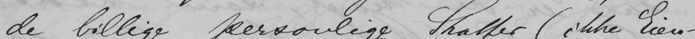de billige personlige Skatter (ikke Eien-
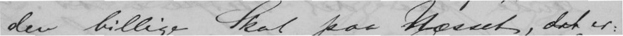den billige Skat paa Næsset, der er:
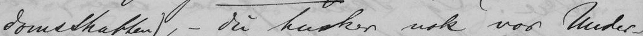domsskatten), - Du husker nok vor Under-
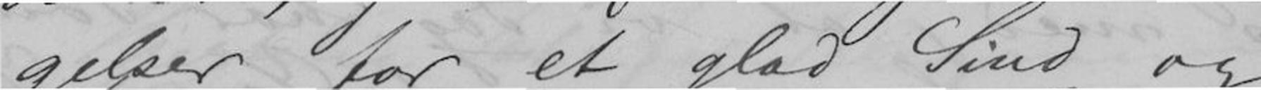gelser for et glad Sind og
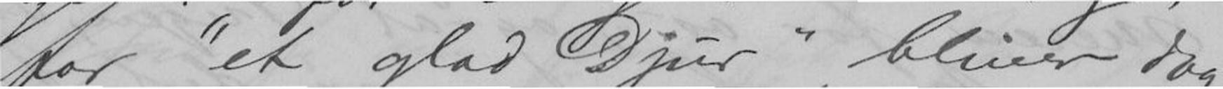for "et glad Djur" bliver dog
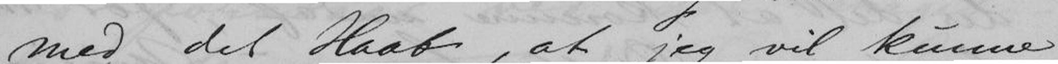med det Haab, at jeg vil kunne
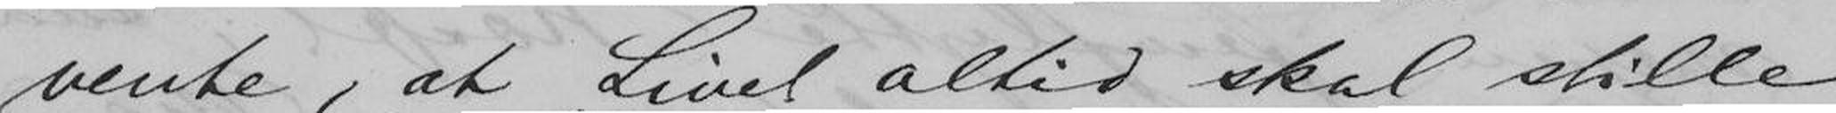vente, at Livet altid skal stille
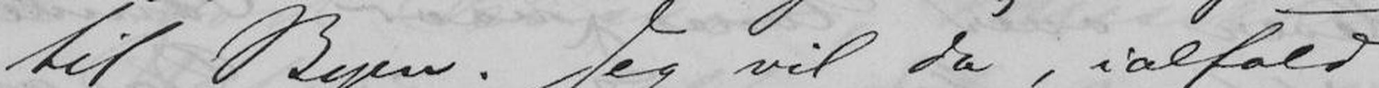til Byen. Jeg vil da, ialtfald
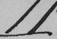II
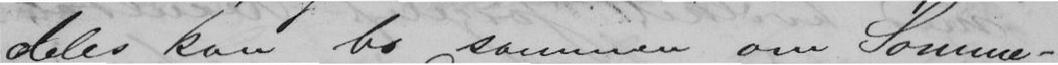deles kan bo sammen om Somme-
